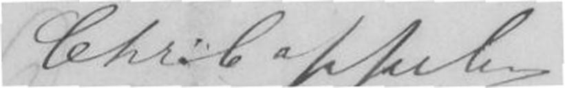Chr. Cappelen
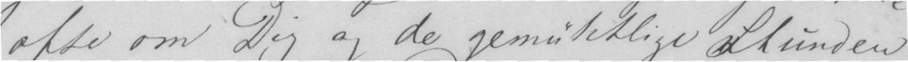ofte om Dig og de gemuttlige Stunden
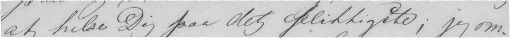at hilse Dig paa det flittigste, jeg om-
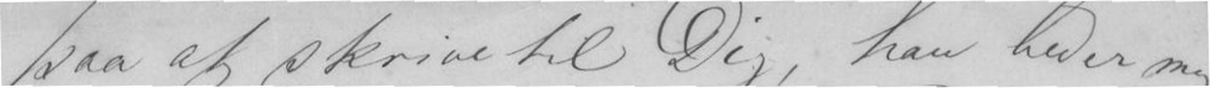paa at skrive til Dig, han beder mig
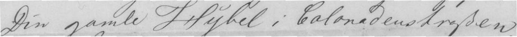Din gamle Hybel i Colonadenstrysen.
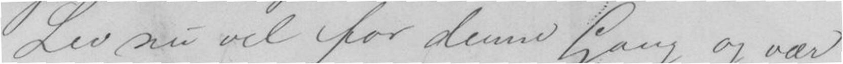Lev nu vel for denne Gang og vær
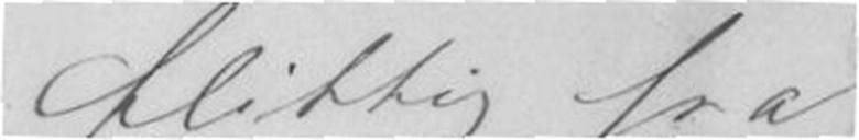flittig fra
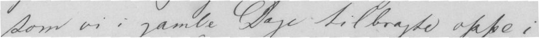som vi i gamle Dage tilbragte ofte oppe i
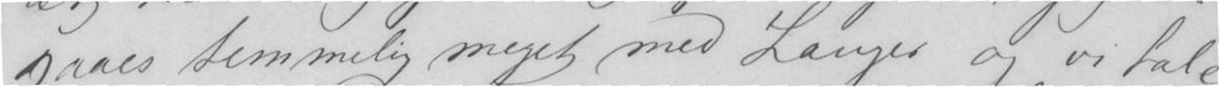gaaes temmelig meget med Langer og vi tale
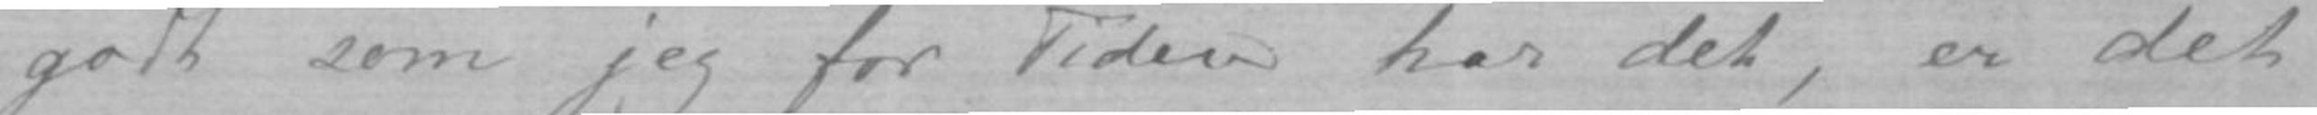godt som jeg for Tiden har det, er det
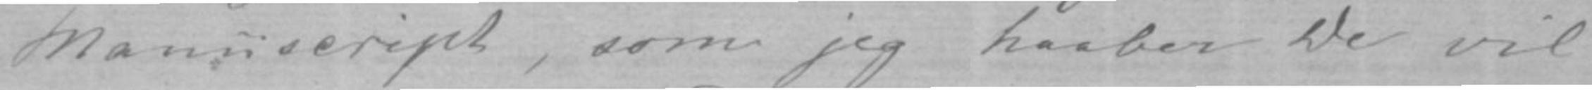Manuscript, som jeg haaber De vil
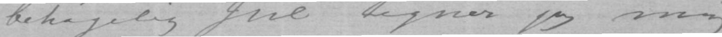behagelig Jul tegner jeg mig
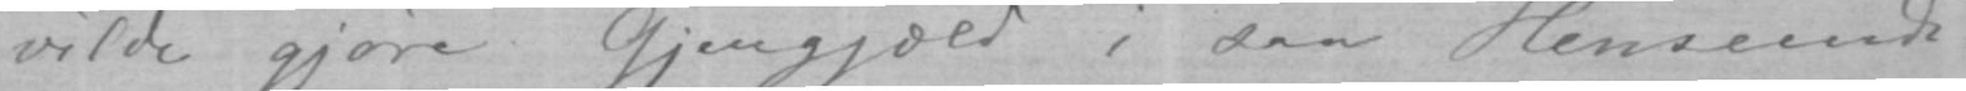vilde gjøre Gjengjæld i saa Henseende.
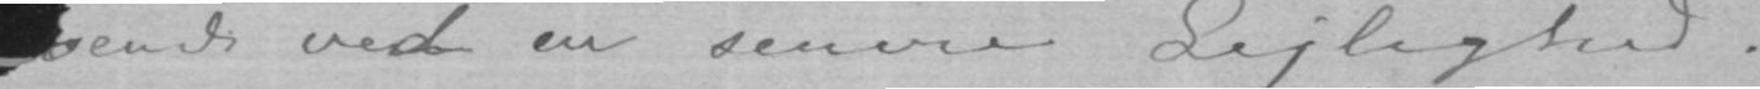sende vedve en senere Lejlighed.
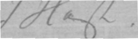I Hast!
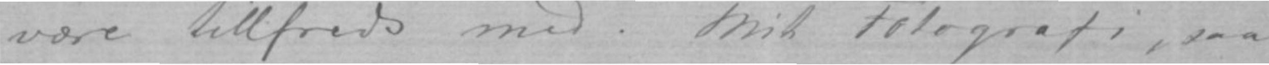være tillfreds med. Mit Fotografi, saa
